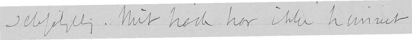selvfølgelig. Mit hode har ikke kunnet
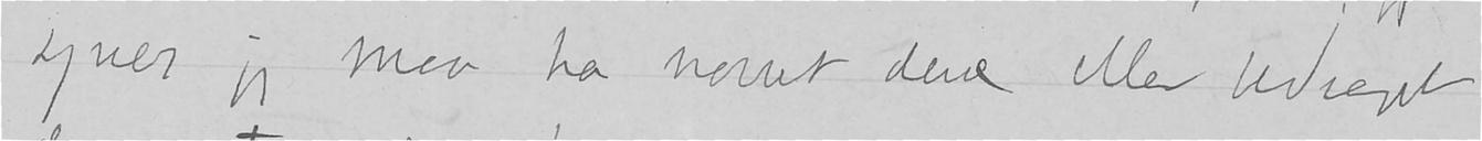synes jeg maa ha nævnt dere eller bedraget
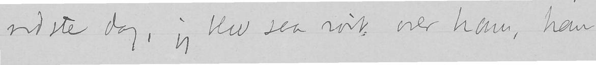sidste dag, jeg blev saa rørt over ham, han
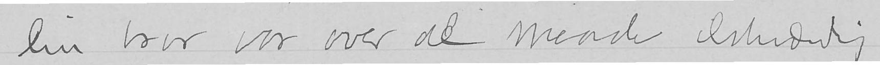Din bror var over al maade elskværdig
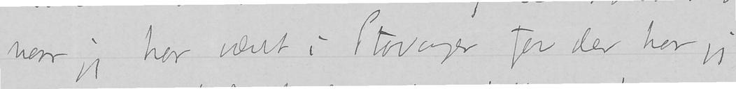naar jeg har været i Stavanger for der har jeg
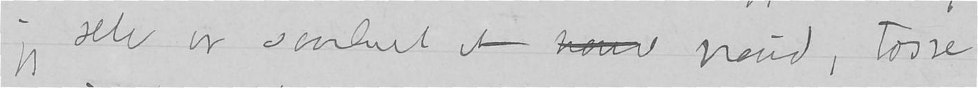jeg selv er saadant et hans neud, tosse
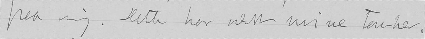paa mig. Dette har været mine tanker,
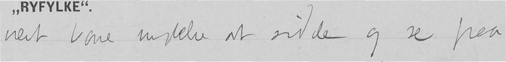vært bare nytelse at sidde og se paa
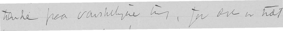tænke paa vanskeligere ting, for ære er lidt
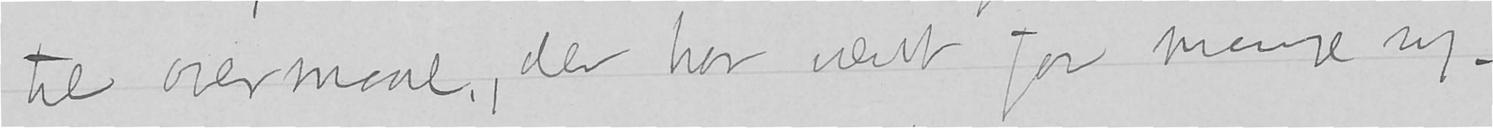til overmaal, der har været for mange eng-
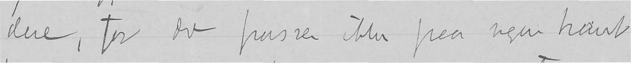dere, for det passer ikke paa nogen kant
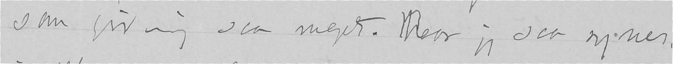som gir mig saa meget. Naar jeg saa synes,
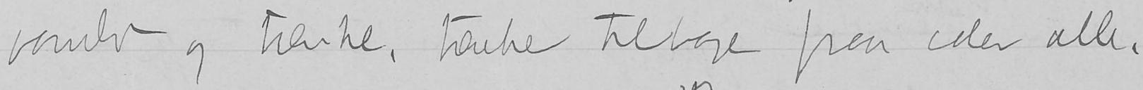vandet og tænke, tænke tilbage paa eder alle,
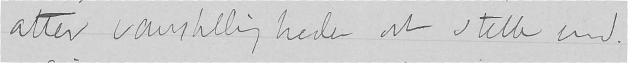atter vanskeligheder at stille med.
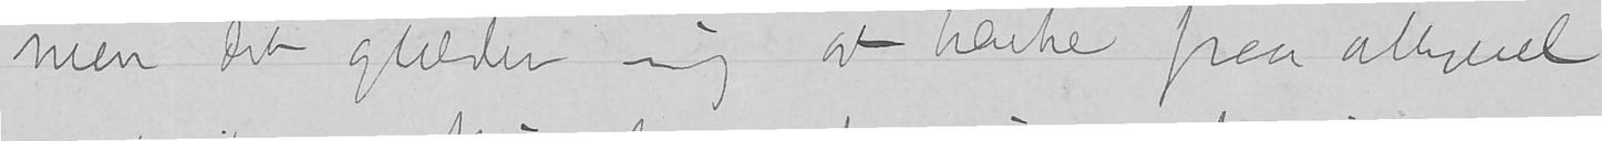men det glæder mig at tænke paa alligevel
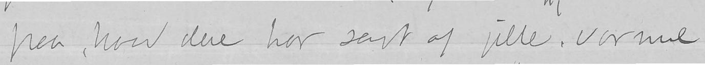paa, hvad dere har sagt af jille, varme
